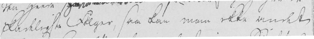skadeligste Følger, saa kan man ikke andet
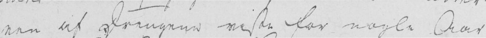een af Drengene viste for nogle Aar
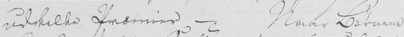uddelte Præmier - Naar Børnene
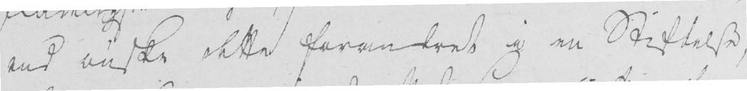end ønske dette forandret i en Stiftelse,
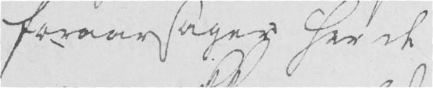foraarsager har de
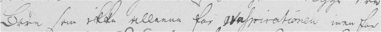Børn som ikke alleene for saspirationen men for
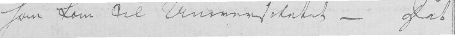han kom til Universitetet - Det
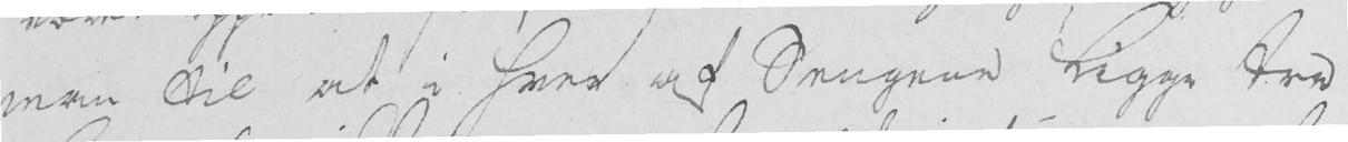man til at i hver af Sengene ligge tre
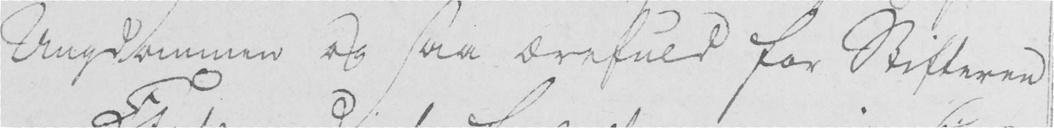Ungdommen og saa ærefuld for Stifteren.
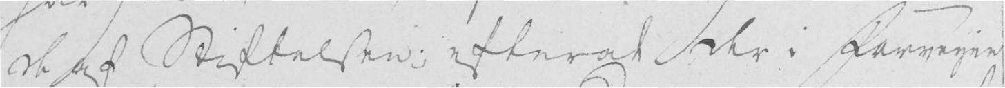de af Stiftelsen; efterat der i Forveyen
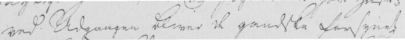ved Udgangen bliver de gandske forsynet
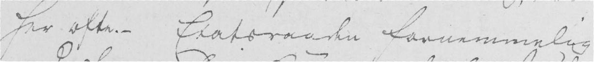her ofte. - Etatsraaden fornemmelig
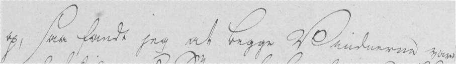op, saa fandt jeg at begge Vinduerne var
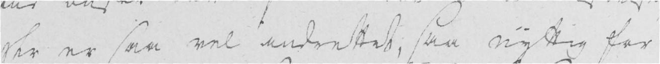der er saa vel andrettet, saa nyttig for
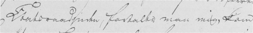Etatsraadinden, fortalte man mig, kom
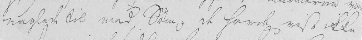naglede til med Søm; de havde vist ikke
